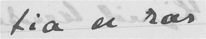tia er rar -.
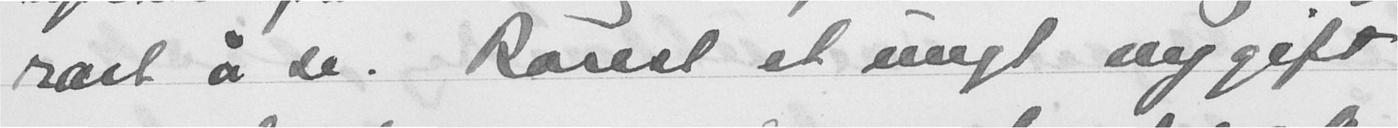rart å se. Rarest et ungt nygift
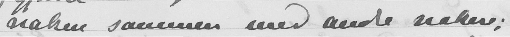naken sammen med andre nakne;
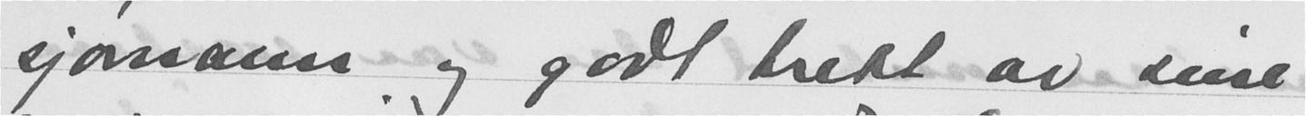sjømann og godt trett av sine
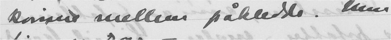kvinnen mellom påkledde. Men
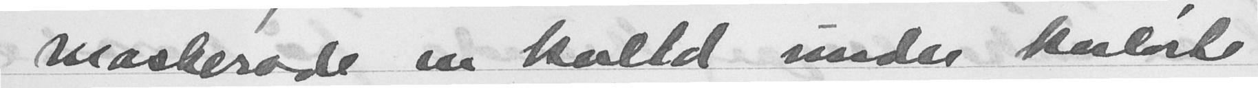maskerade en kveld under kulørte
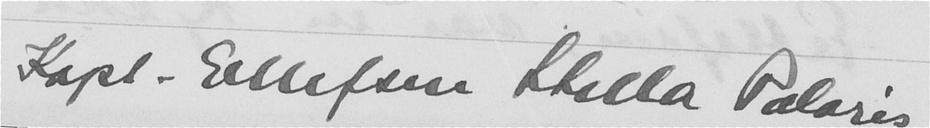Kapt. Ellefsen Stella Polaris
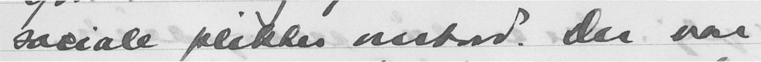sociale plikter ombord. Der var
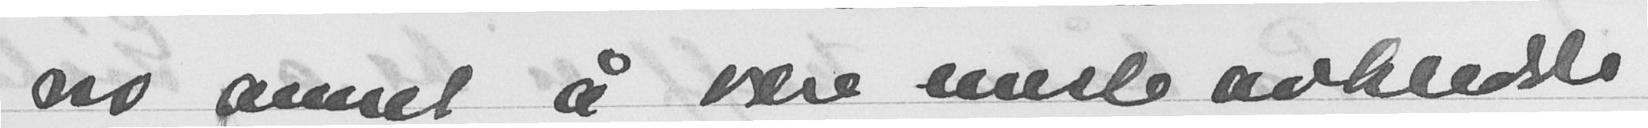no annet å være meste avkledde
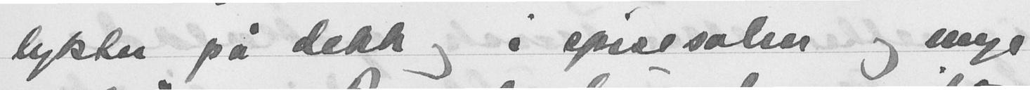lykten på dekk og i spisesalen og mye
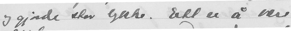og gjorde stor lykke. Ett er å være
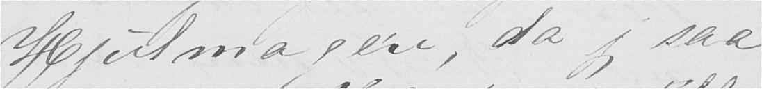Hjulmagere, da jeg saa
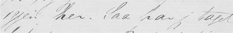igjen her. Saa har jeg taget
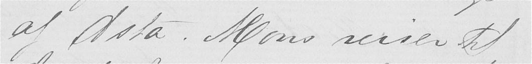af Asta. Mons reiser til
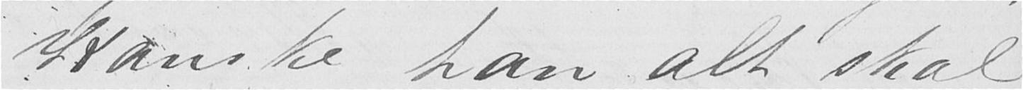Kanske han alt skal
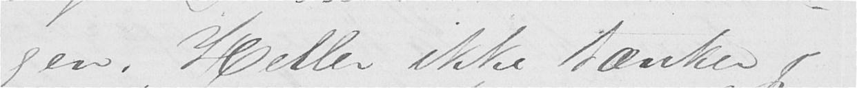gen. Heller ikke tænker jeg
